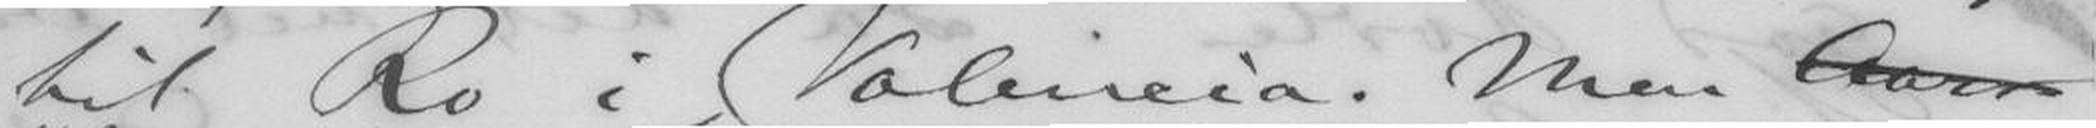til Ro i Valencia. Men Aars-
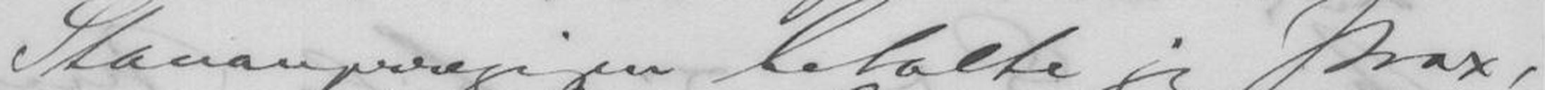Stavangerregningen betalte jeg strax,
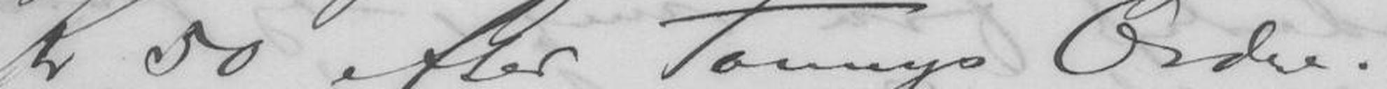Kr 50 efter Tonnys Ordre. -
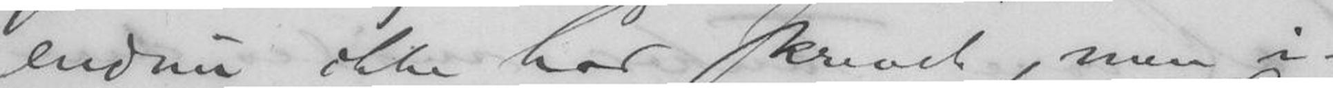endnu ikke har skrevet, men i-
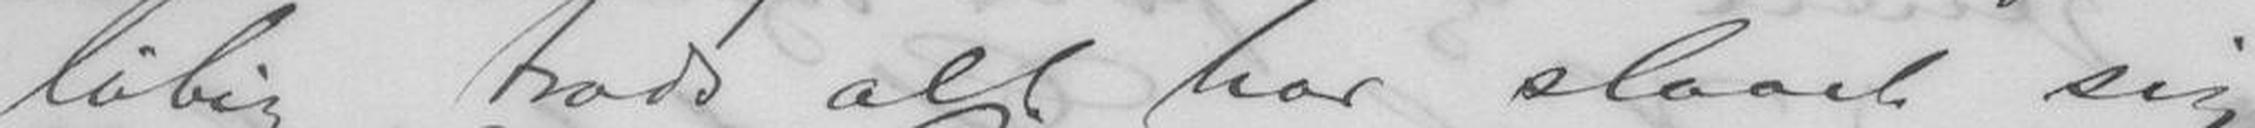løbig trods alt har slaaet sig
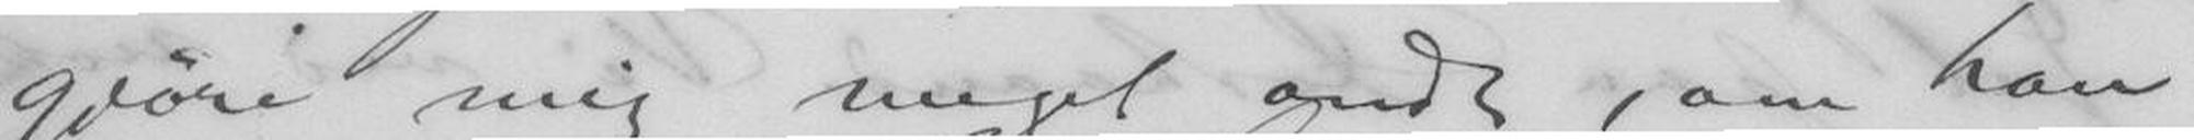gjøre mig meget ondt, om han
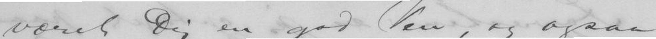været Dig en god Ven, og ogsaa
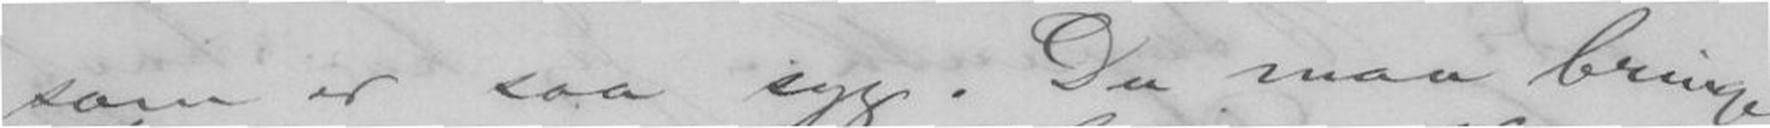som er saa syg. Du maa bringe
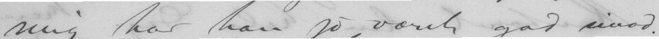mig har han jo været god imod.
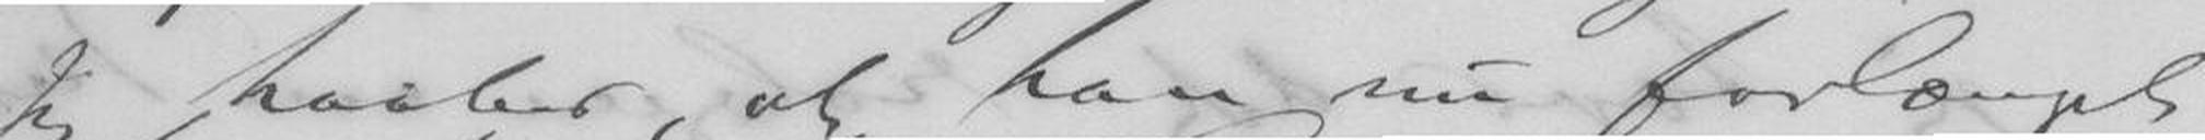Jeg haaber, at han nu forlængst
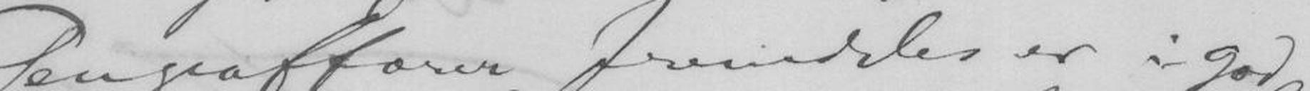Pengeaffærer fremdeles er i god
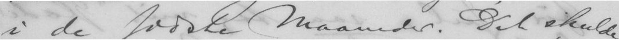i de sidste Maaneder. Det skulde
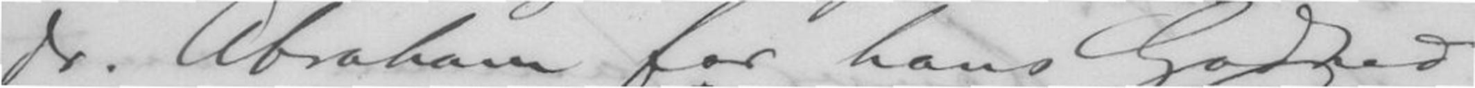Dr. Abraham for hans Godhed
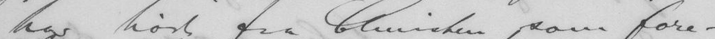har hørt fra Christen som fore-
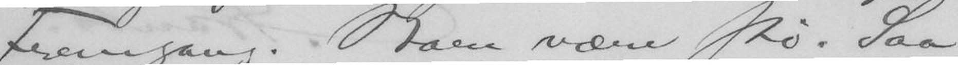Fremgang. Bare være stø. Saa
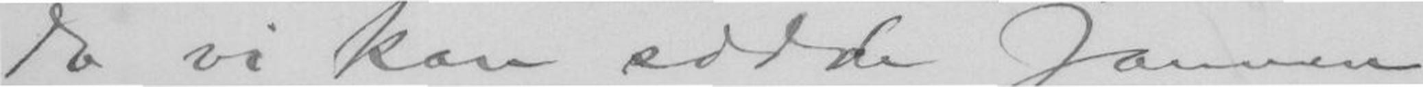da vi kan sidde sammen
